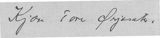Kjære Tore Ørjasæter,
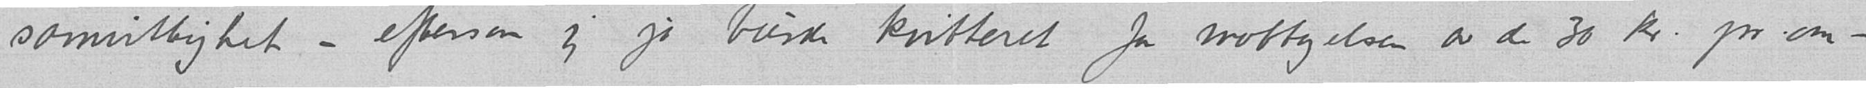samvittighet – eftersom jeg jo burde kvitteret for mottagelsen av de 30 kr. pr. om-
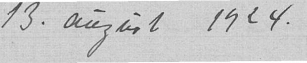13. august 1924.
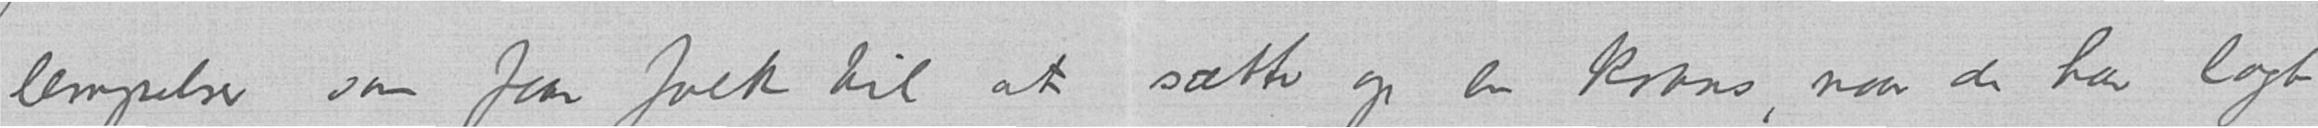lempelser som faar folk til at sætte op en krans, naar de har lagt
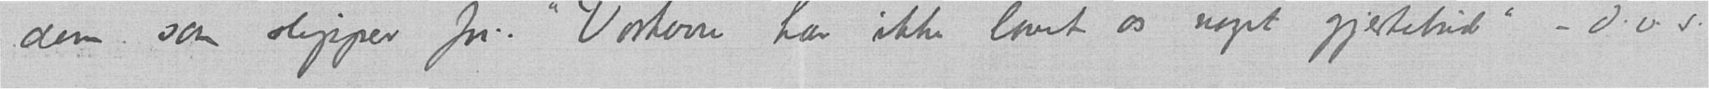dem som slipper fri. "Vorherre har ikke lovet os noget gjestebud" – d.v.s.
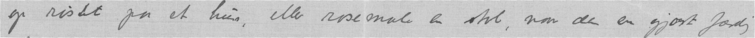op ristet paa et hus, eller rosemale en stol, naar den en gjort færdig
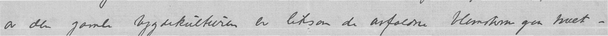av den gamle bygdekulturen er liksom de avfaldne blomsterne paa træet –
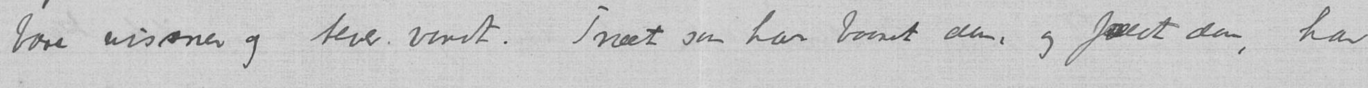bare vissner og tever vondt. Træet som har baaret dem, og fældt dem, har
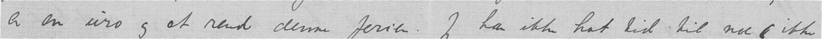er en uro og et rend denne ferien. Jeg har ikke hat tid til noe (ikke
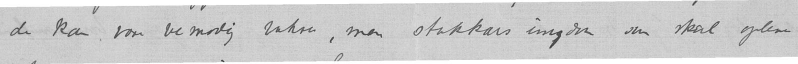de kan være vemodig vakre, men stakkars ungdom som skal opleve
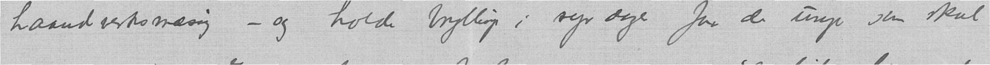haandverksmæsig – og holde bryllup i syv dager for de unge som skal
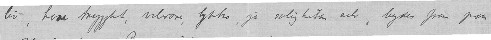liv, hvor trygghet, velvære, lykke, ja saligheten selv, bydes frem paa
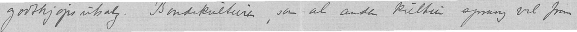godtkjøpsutsalg. Bondekulturen, som al anden kultur sprang vel frem
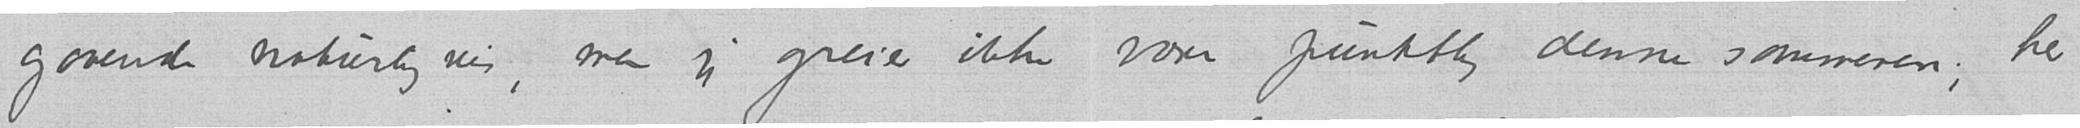gaaende naturligvis, men jeg greier ikke være punktlig denne sommeren; her
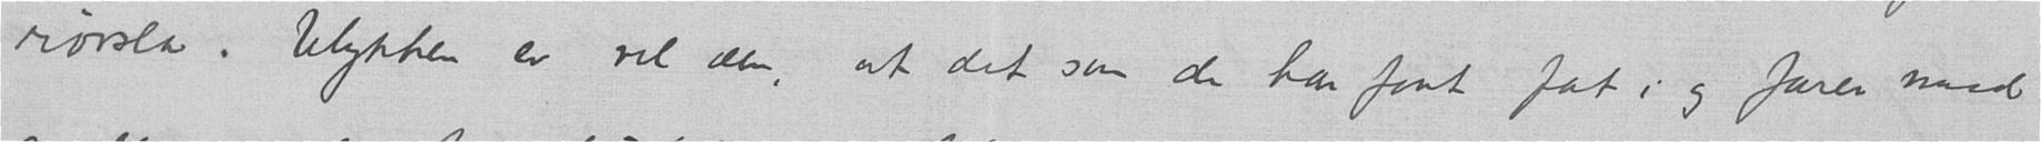rørsla. Ulykken er vel den, at det som de har faat fat i og farer med
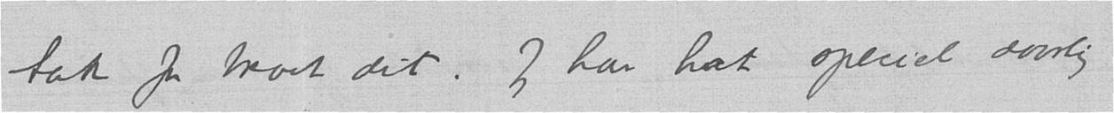tak for brevet dit. Jeg har hat speciel daarlig
